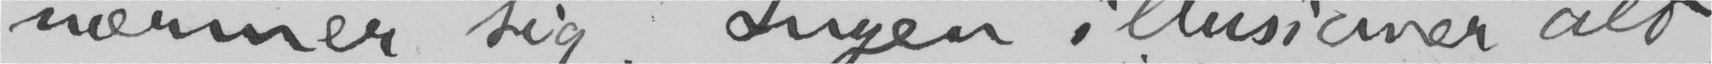nærmer sig. Ingen illusioner alt-
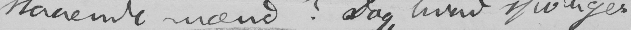staaende mænd? Dog, hvad spørger
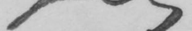jeg
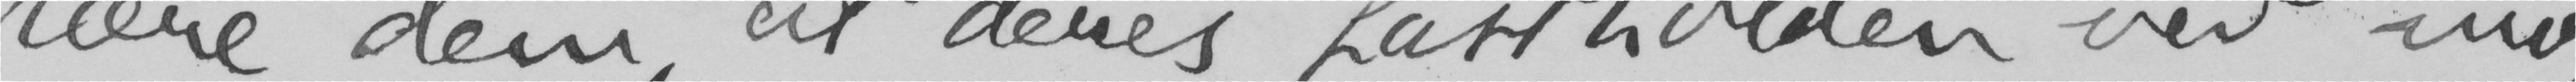lære dem, at deres fastholden ved mo-
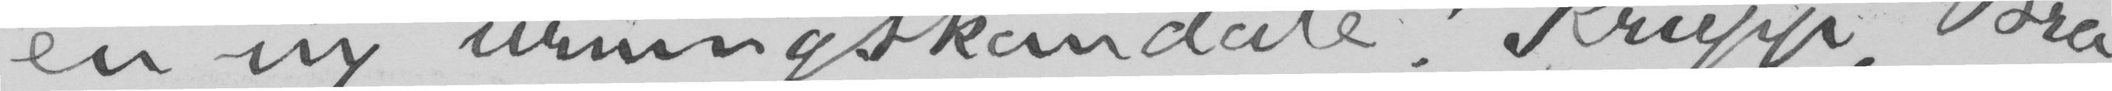en ny urningskandale! Krupp, Bra-
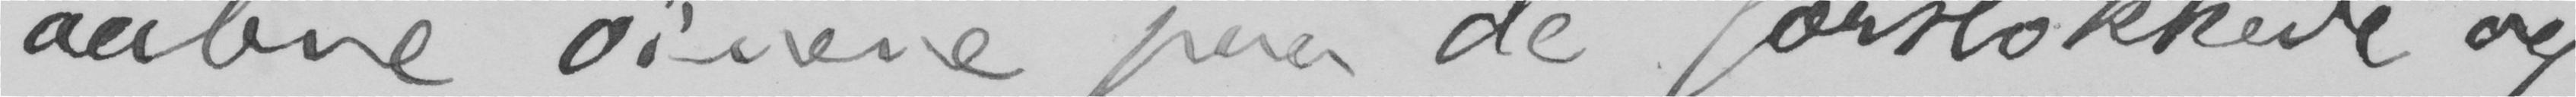aabne øinene paa de forstokkede og
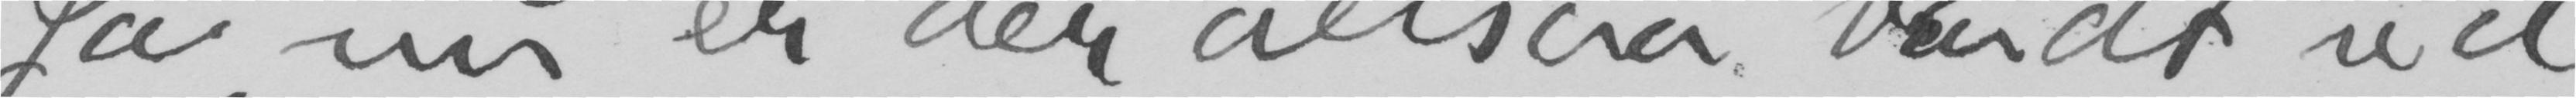Ja, nu er der altsaa brudt ud
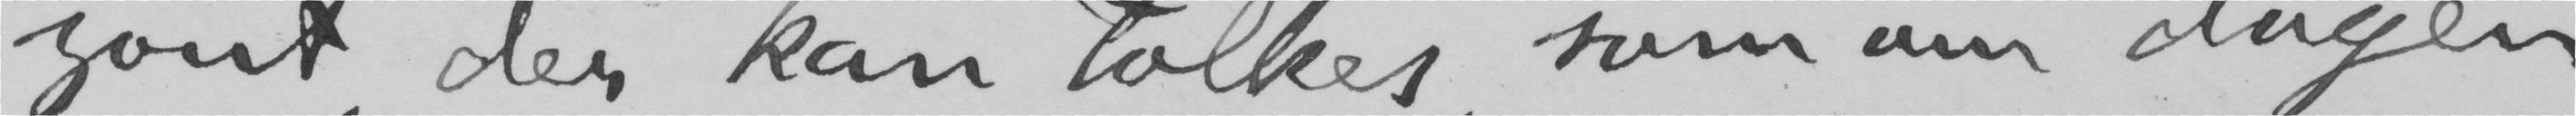sont, der kan tolkes, som om dagen
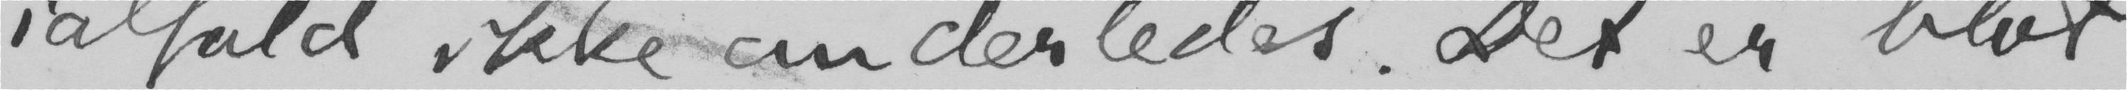ialfald ikke anderledes. Det er blot
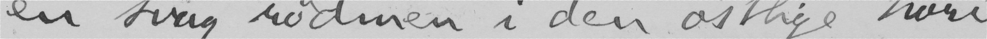en svag rødmen i den østlige hori-
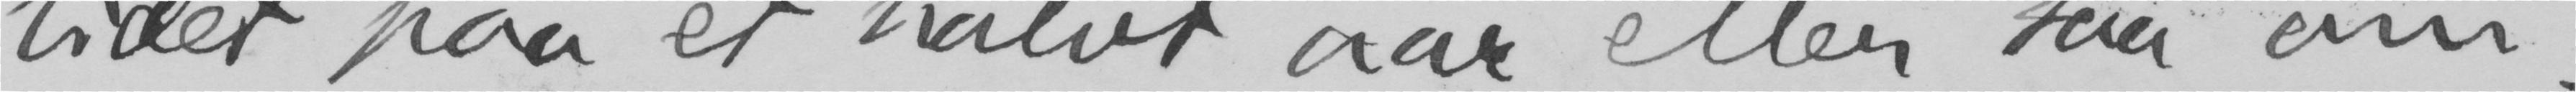lidet paa et halvt aar eller saa om-
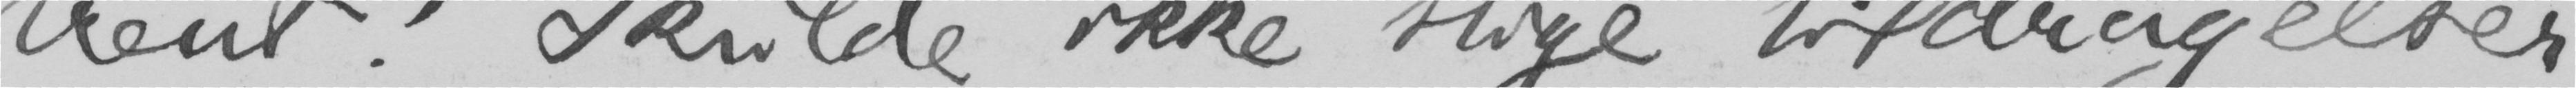trent! Skulde ikke slige tildragelser
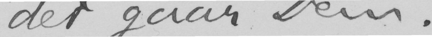det gaar Dem.
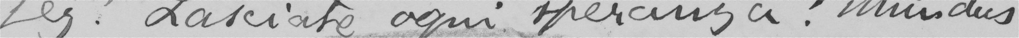jeg! Lasciate ogni speranza! Mundus
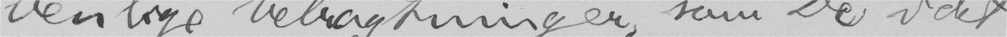venlige betragtninger, som De i det
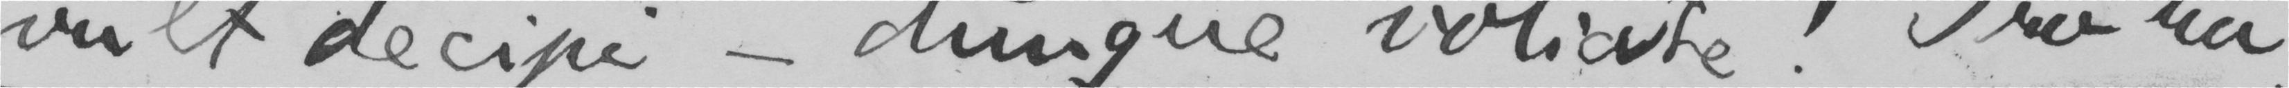vult decipi, – dunque volaste! Pro ra-
